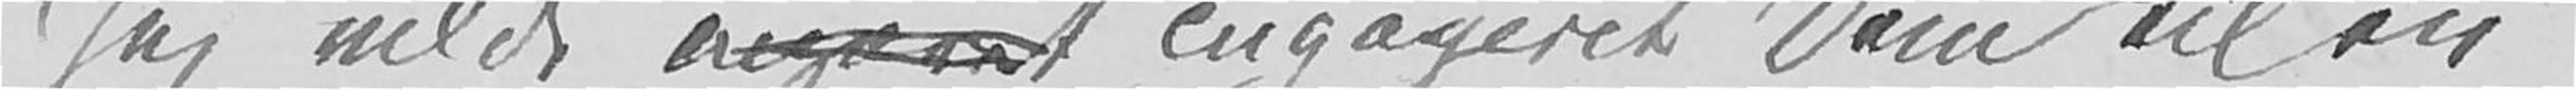Jeg vilde enga ret engageret Dem til en
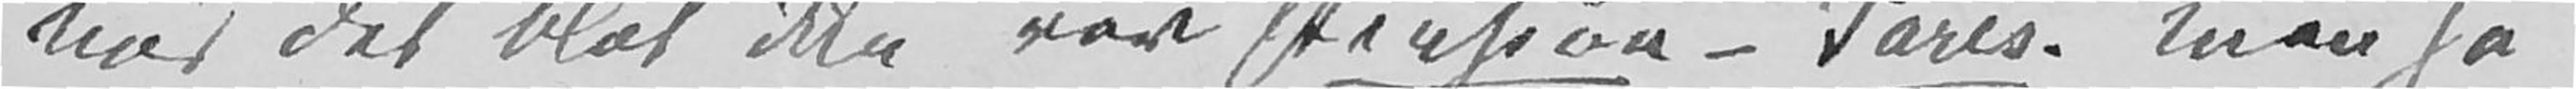når det blot ikke var Pension-Paris, men så
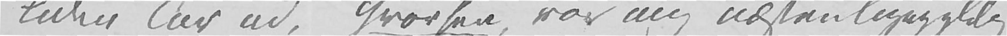liden Tur ud, hvorhen, var mig næsten ligegyldig
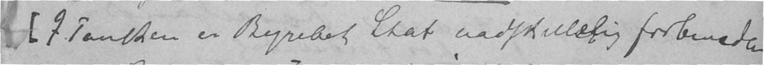[I Tanken er Begrebet Stat uadskillig forbunden
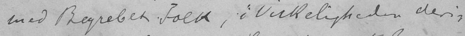med Begrebet Folk, i Virkeligheden deri-
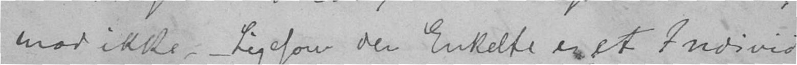mod ikke. Ligesom den Enkelte er et Individ
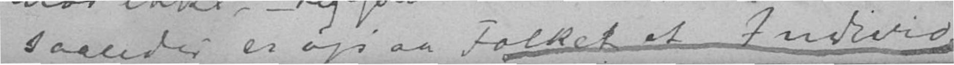saaledes er ogsaa Folket et Individ
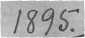1895.
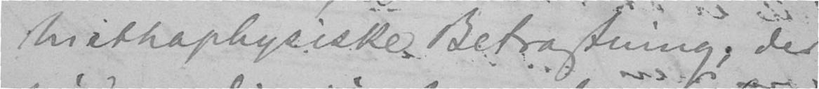metaphysiske Betragtning, der
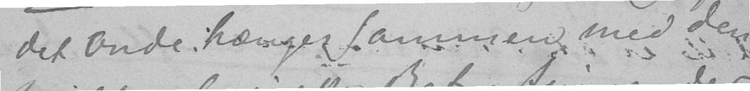det Onde hænger sammen med den
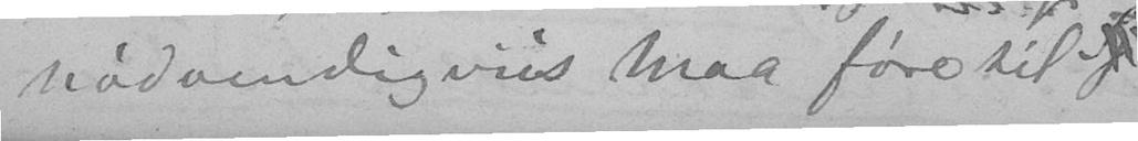nødvendigvis maa føre til
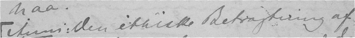[Anm: den ethiske Betragtning af
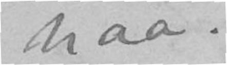naa.
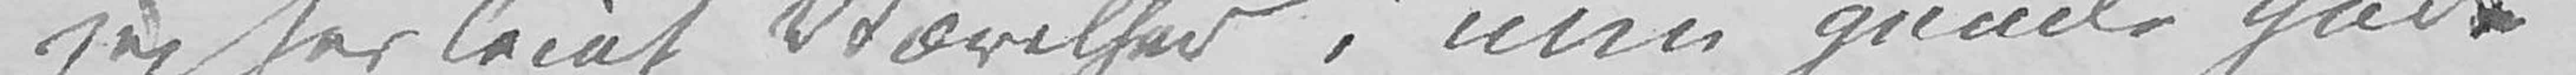jeg har leiet Værelser i min gamle gode
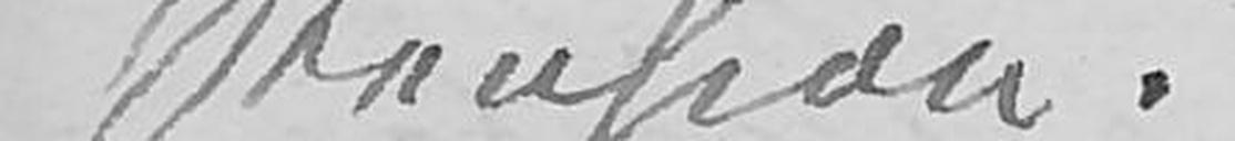Skal vi ikke sees forinden?
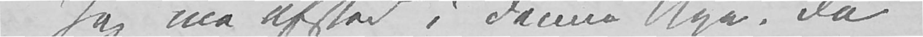Jeg må afsted i denne Uge, da
